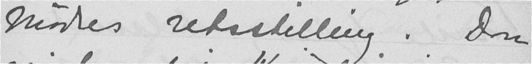mødres retsstilling. Don
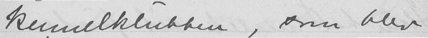kennelklubben, som blev
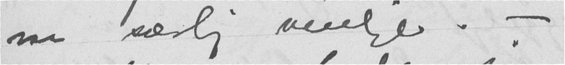var særlig venlige. -
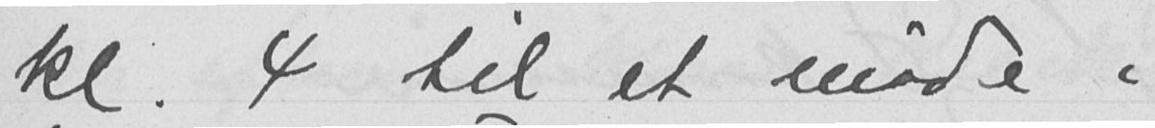kl. 4 til et møde i
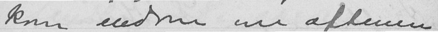kom indom om aftenen
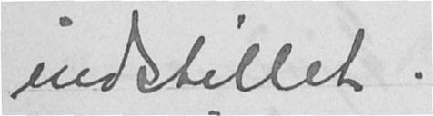indstillet.
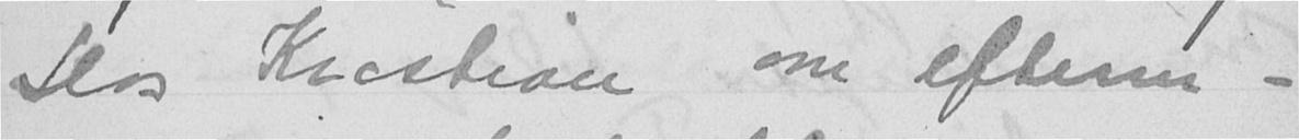Hos Kristian om efterm -
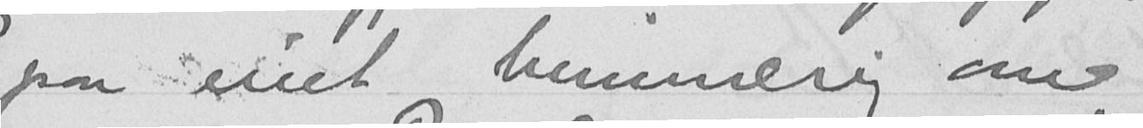paa mit himmerig om
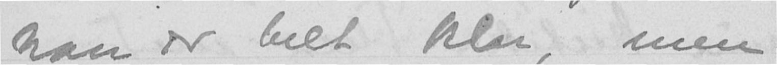han er helt klar, men
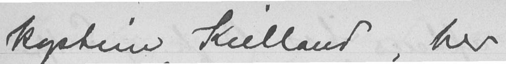kaptein Kielland, her
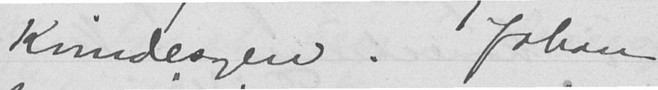Kvindesagen. Johan
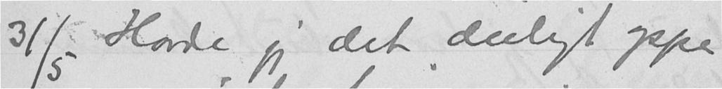31/5 Havde jeg det deiligt oppe
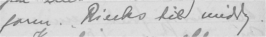form. Riecks til middag.
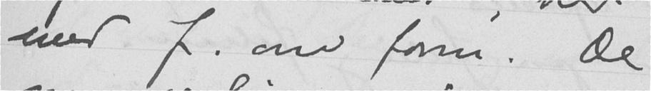med J. om form. De
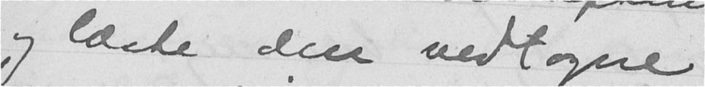og læste den vedtagne
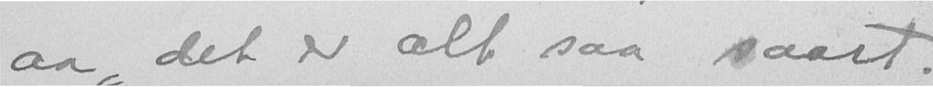aa, det er alt saa saart.
